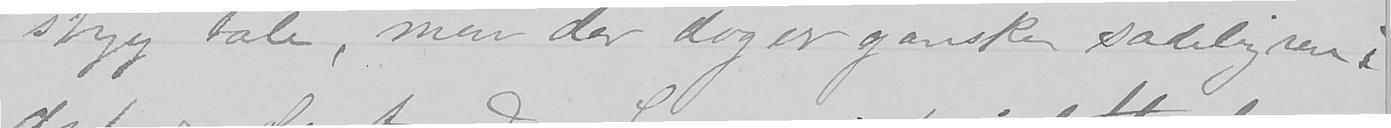styg tale, men der doger ganske sædelig ren i
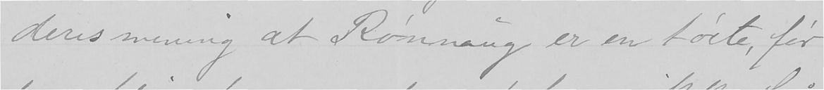deres mening at Rønnang er en tøite, før
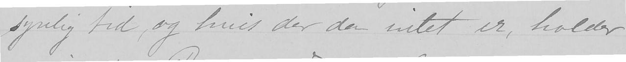synlig tid, og hvis der da intet er, holder
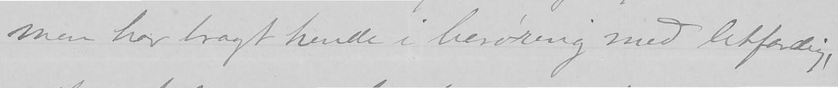men har bragt hende i berøring med letfærdig,
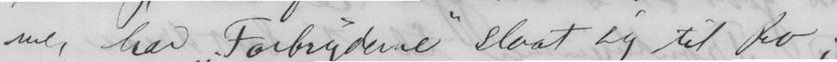me, har Forbruderne slaat sig til Ro;
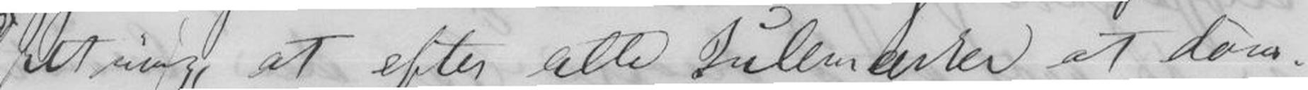retning, at efter alle Julemærker at døm-
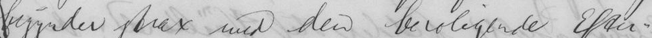begynder strax med den beroligende Efter-
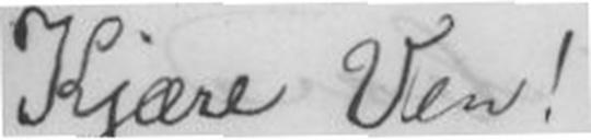Kjære Ven!
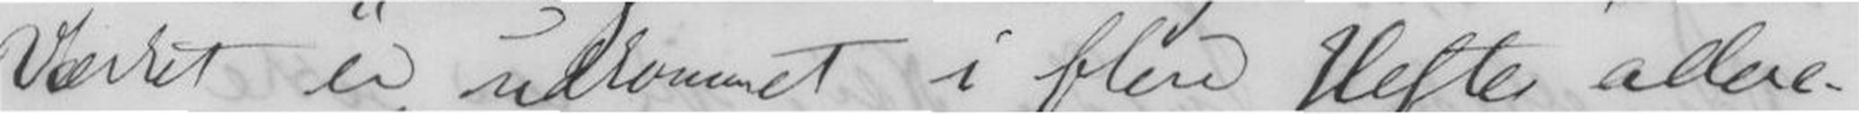Værket er udkommet i flere Hefte allere-
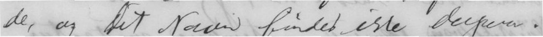de, og Dit Navn findes ikke derpaa.
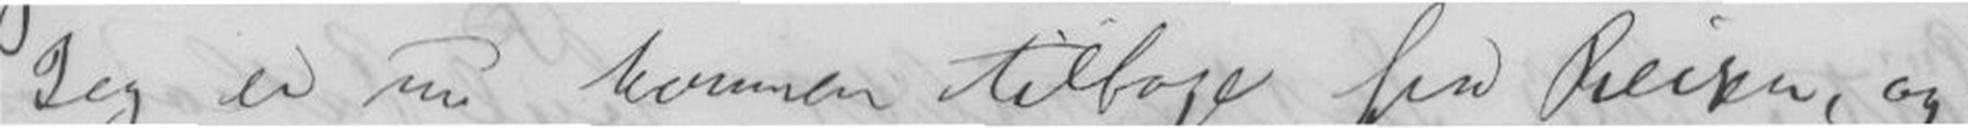Jeg er nu kommen tilbage fra Reisen, og
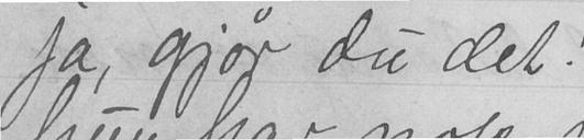ja, gjør du det!
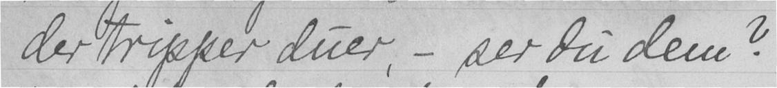der tripper duer, - ser du dem?
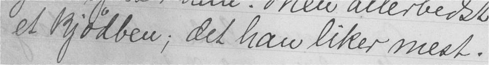et kjødben; det han liker mest.
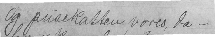Og pusekatten vores, da -
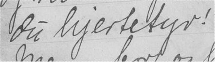du hjertetyv!
